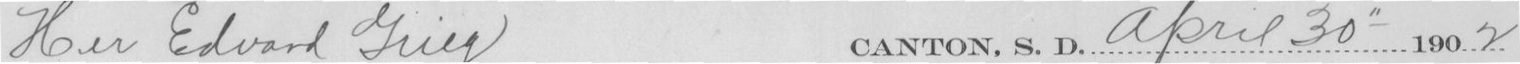Her Edvard Grieg Canton, S. D. April 30" 1902
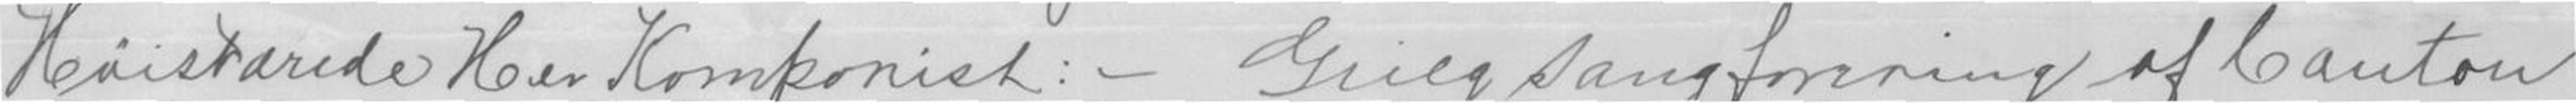Høistærede Her Komponist: - Grieg Sangforening af Canton
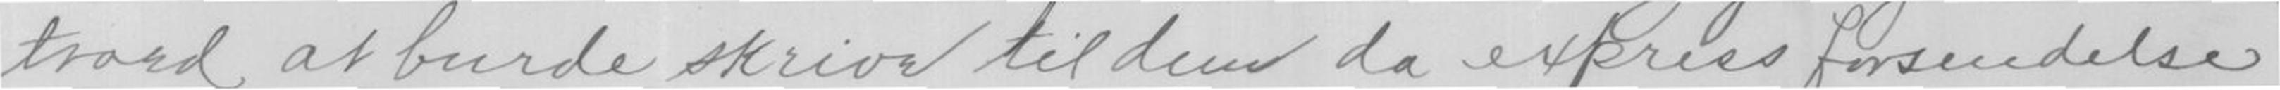troed at burde skrive til dem da express forsendelse
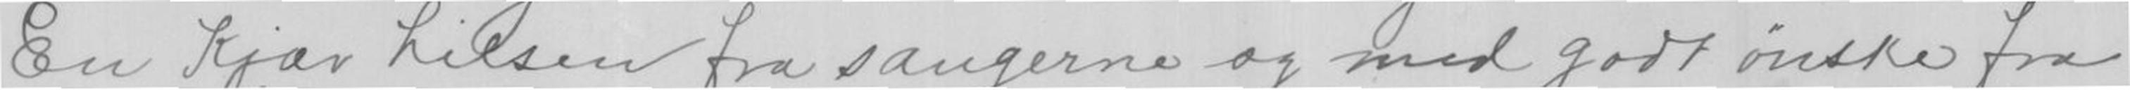En Kjær hilsen fra sangerne og med godt ønske fra
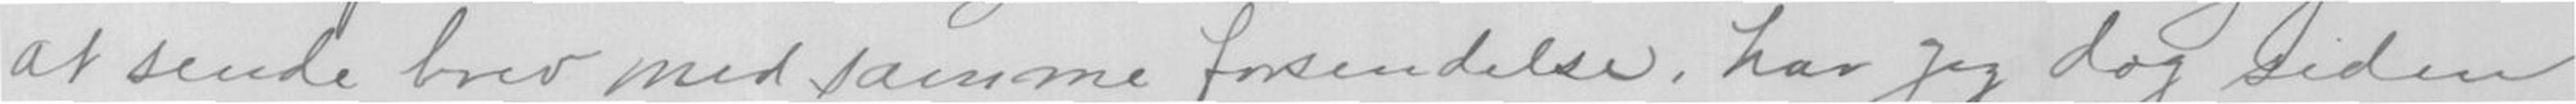at sende brev med samme forsendelse, har jeg dog siden
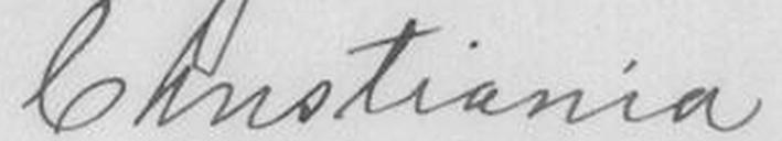Christiania
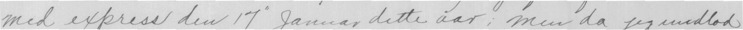med express den 17" Januar dette aar; men da jeg undlod,
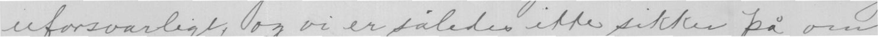uforsvarligt, og vi er således ikke sikker på om
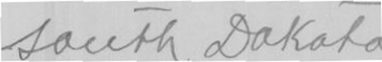South Dakota
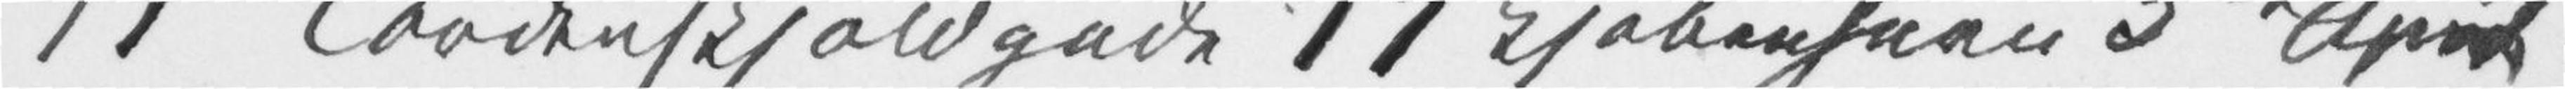17 Tordenskjoldgade 17 Kjøbenhavn 3de April
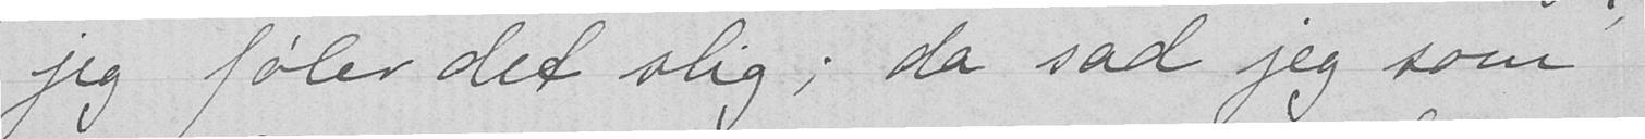jeg føler det slig; da sad jeg som
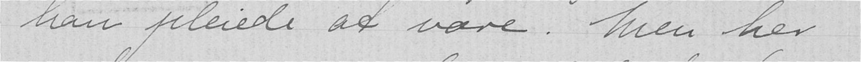han pleiede at være. Men her
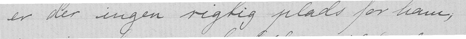er der ingen rigtig plads for ham;
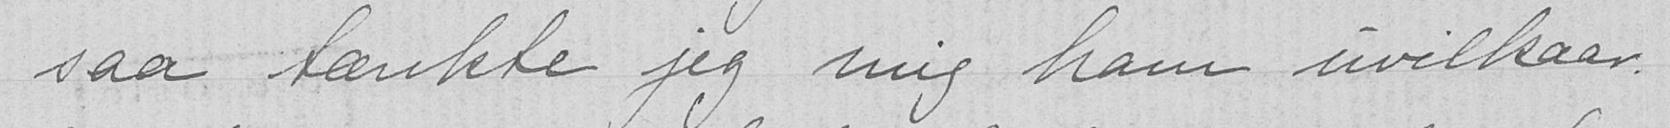- saa tænkte jeg mig ham uvilkaar-
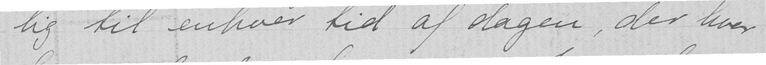lig til enhver tid af dagen, der hvor
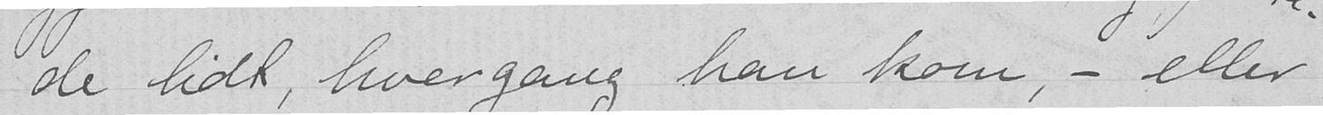de lidt, hvergang han kom, - eller
